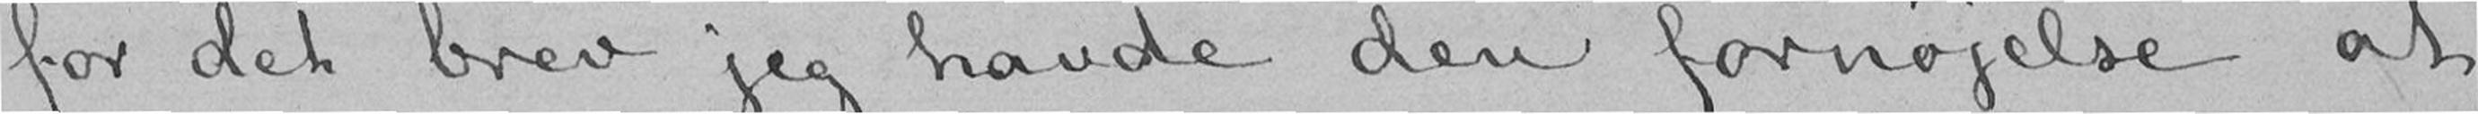for det brev jeg havde den fornøjelse at
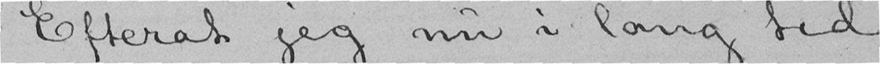Efterat jeg nu i lang tid
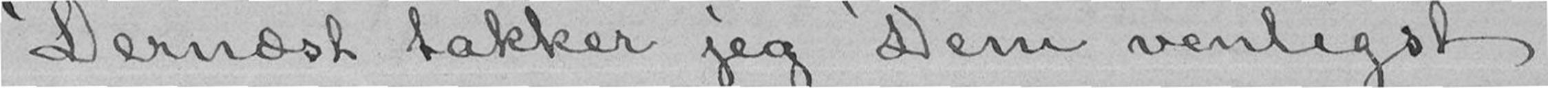Dernæst takker jeg Dem venligst
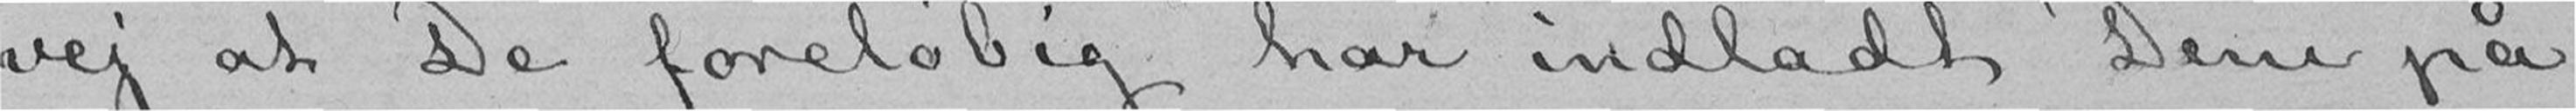vej at De foreløbig har indladt Dem på
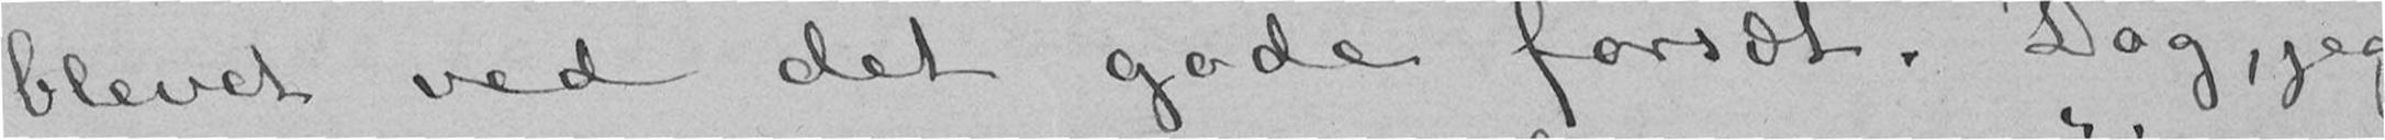blevet ved det gode forsæt. Dog, jeg
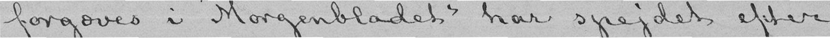forgæves i «Morgenbladet» har spejdet efter
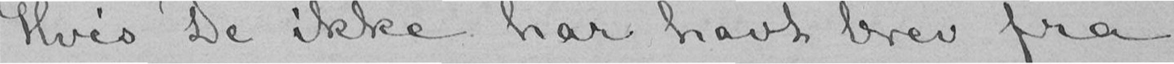Hvis De ikke har havt brev fra
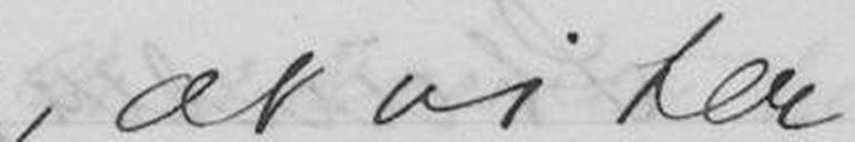, at vi her
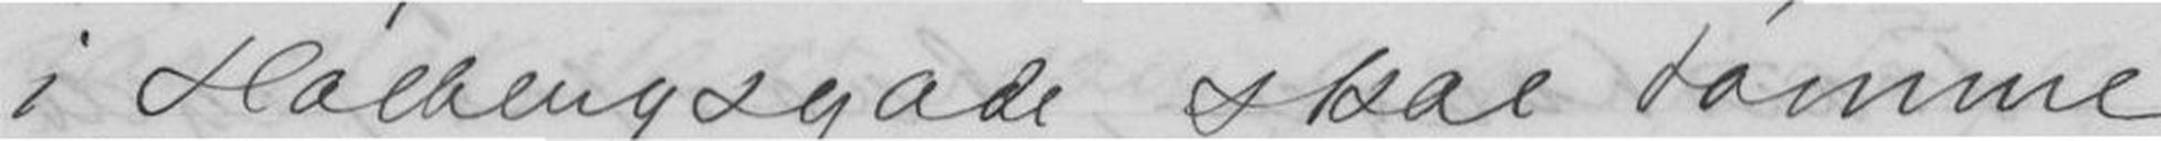i Holbergsgade skal tømme
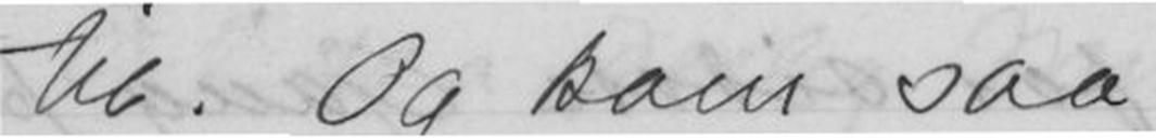til. Og kan saa
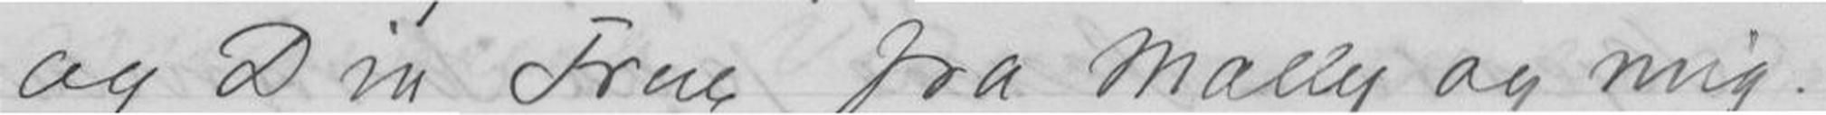og Din Frue fra Mally og mig
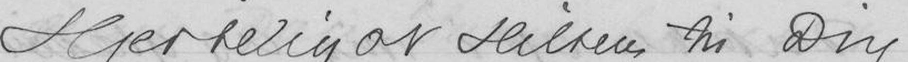Hjerteligst Hilsen til Dig
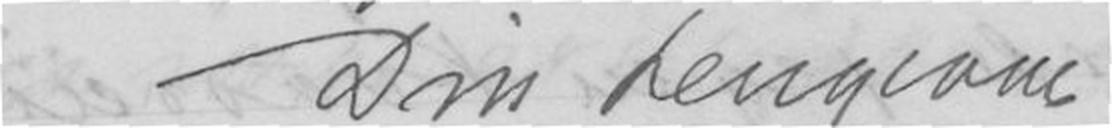Din hengivne
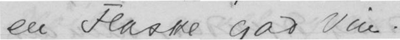en Flaske god vin.
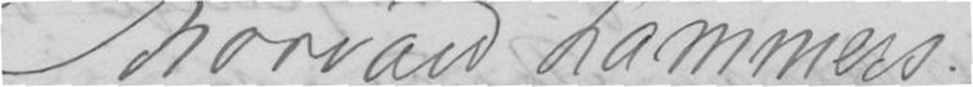Thorvald Lammers.
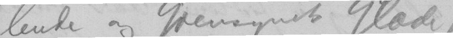lende og Gjensynets Glæde
